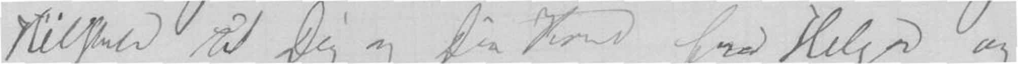Hilsner til Dig og Din Kone fra Helga og
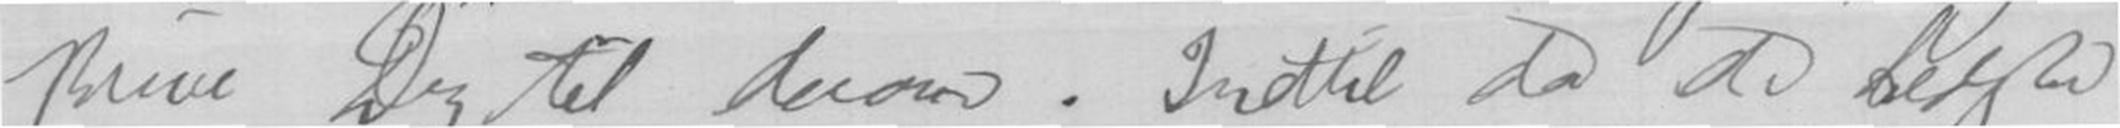skrive Dig til derom. Indtil da de bedste
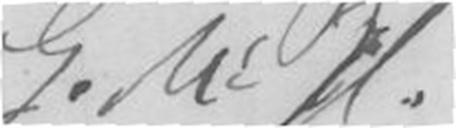G. M=H.
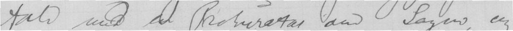tale med en Prokurator om Sagen, og
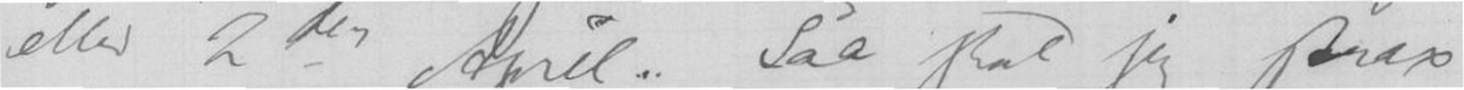eller 2den April. Saa skal jeg strax
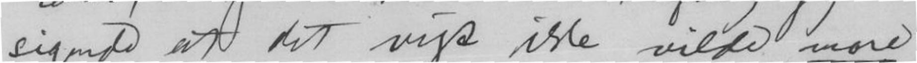sigende at det vist ikke vilde more
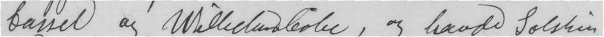Carrel og Willerlunsbøbe, og havde Solskin
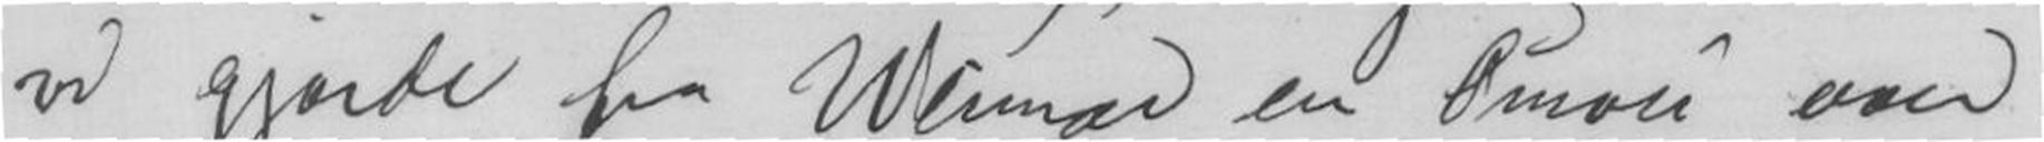vi gjorde fra Weimar en Cmoll over
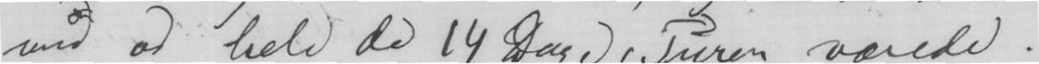med os hele de 14 Dage, Turen værede.
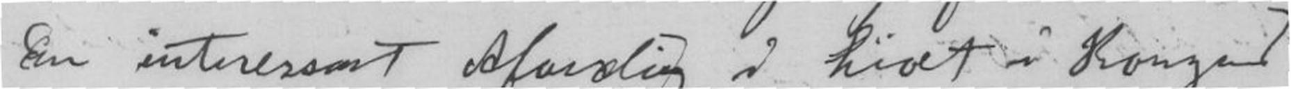En interessant Afvexling i Livet i Kongens
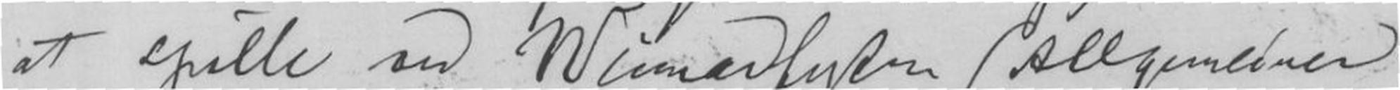at spille ved Wiemarfesten (Allgemeiner
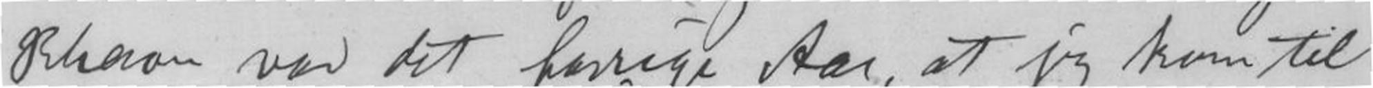Khavn var det forrige Aar, at jeg kom til
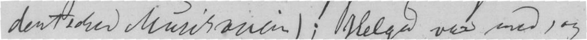deutscher Musikverein); Helga var med, og
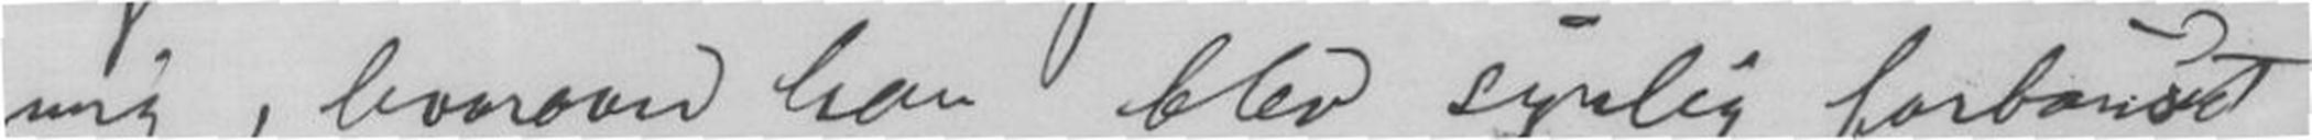mig, hvorover han blev synlig forbauset.
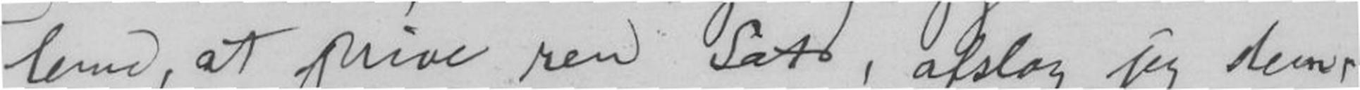lerne, at skrive ren Sats, afslog jeg dem,
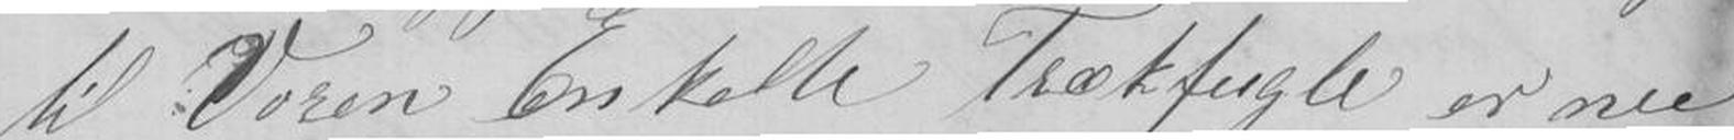til Voren Enkelte Trækfugle er nu
